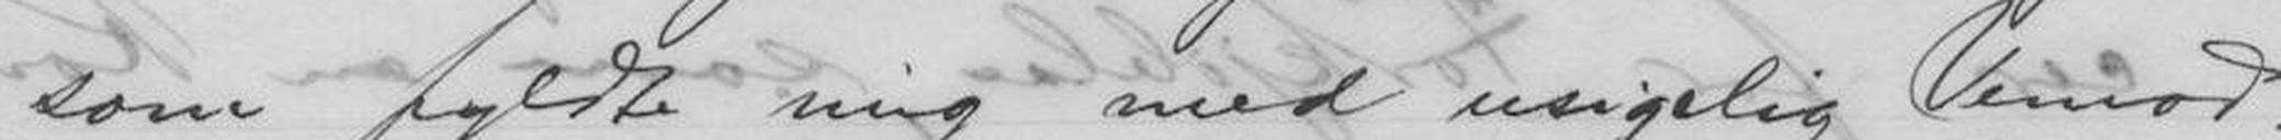som fyldte mig med usigelig Vemod.
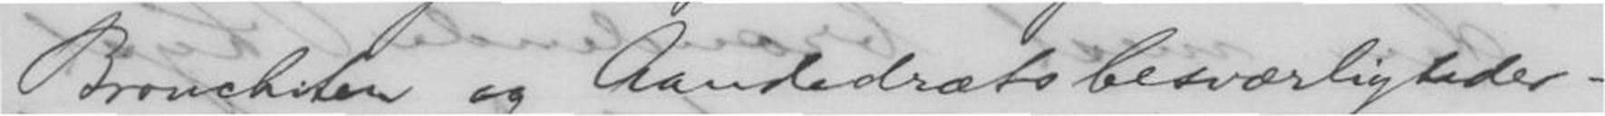Bronchiten og Aanderætsbesværligheder-
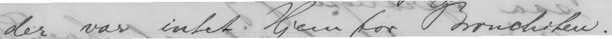der var intet Hjem for Bronchiten.
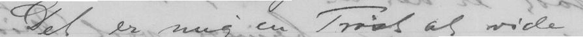Det er mig en Trøst at vide
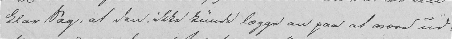klar Sag, at den ikke kunde lægge an paa at være ud-
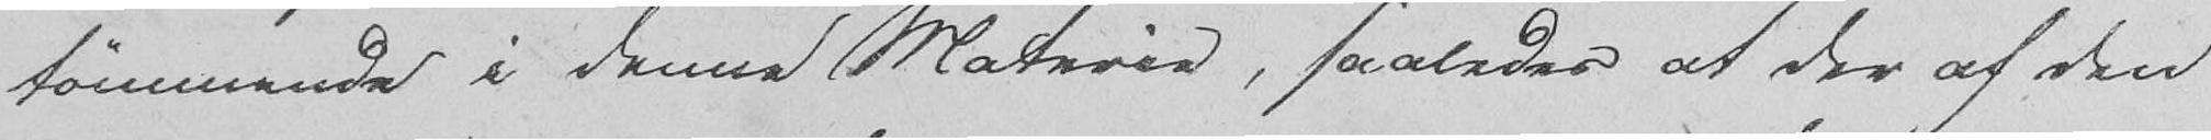tømmende i denne Materie, saaledes at der af den
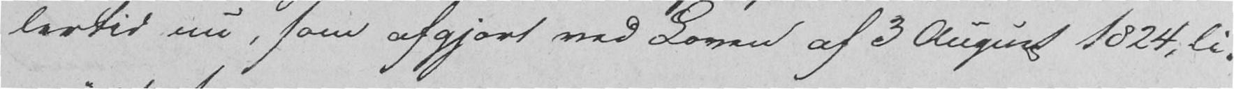lertid nu, som afgjort ved Loven af 3 August 1824, li-
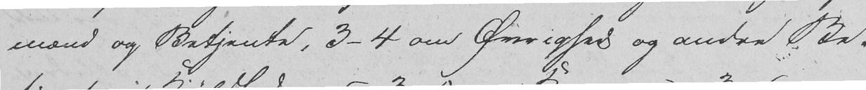mænd og Betjente, 3-4 om Øvrighed og andre Be-
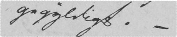gegyldigt. -
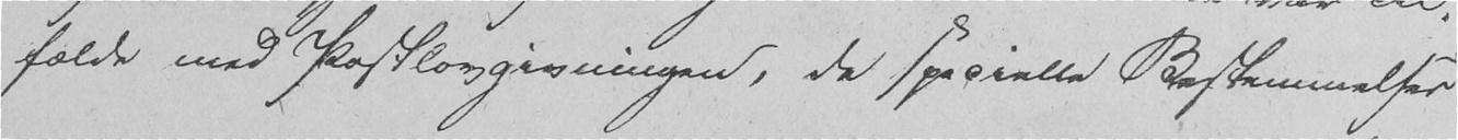fælde med Postlovgivningen, de specielle Bestemmelser
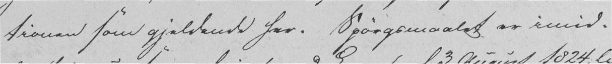tionen som gjeldende her. Spørgsmaalet er imid-
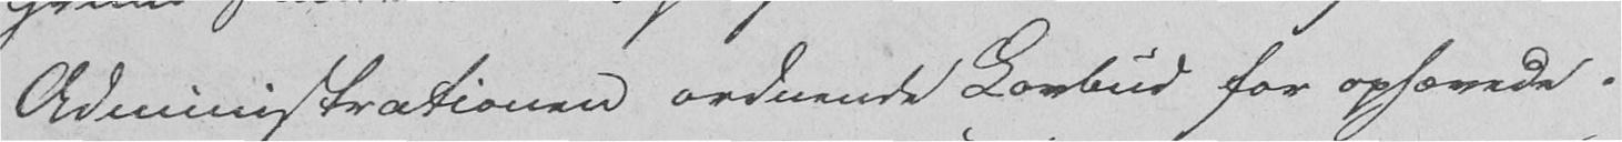Administrationen ordnende Lovbud for ophævede.
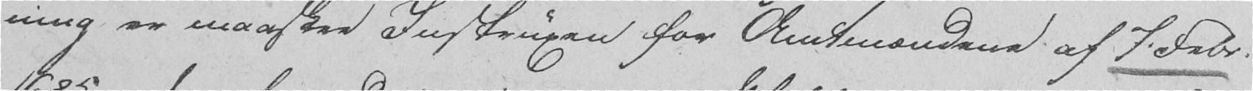ning er maaskee Instruxen for Amtmændene af 7. Febr.
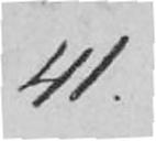41.
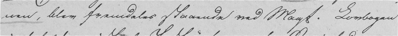nen, blev fremdeles staaende ved Magt. Lovbogen
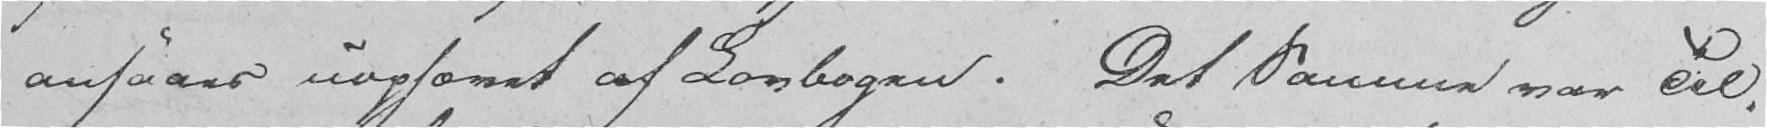ansaaes uophævet af Lovbogen. Det Samme var Til-
